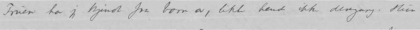Fruen har jeg kjendt fra barn av, likte hende ikke dengang. Hun
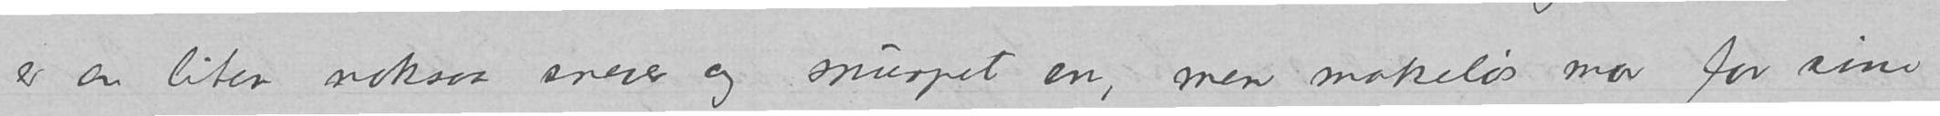er en liten noksaa snever og snurpet en, men makeløs mor for sine
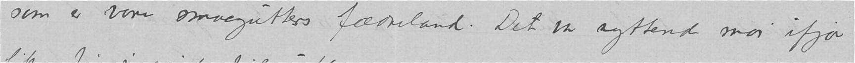som er vore smaagutters fædreland. Det vaer syttende mai ifjor
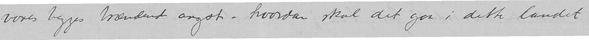vores begges brændende angst – hvordan skal det gaa i dette landet
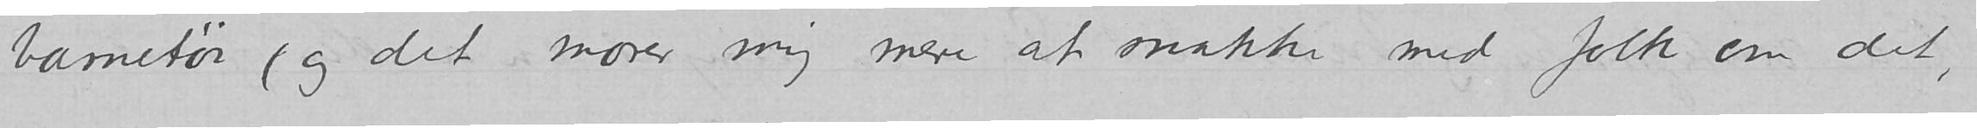barnetøi (og det morer mig mere at snakke med folk om det,
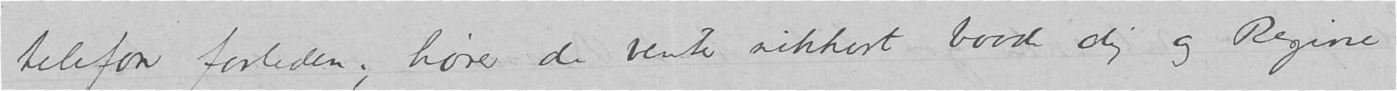telefon forleden; hører de venter sikkert baade dig og Regine
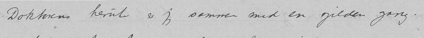Doktoren herute er jeg sammen med en sjelden gang.
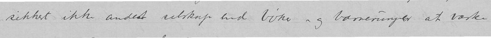sikkert ikke andet selskap end bøker – og barnerumper at vaske.
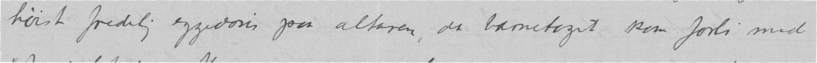høist fredelig eggedosis paa altanen da barnetoget kom forbi med
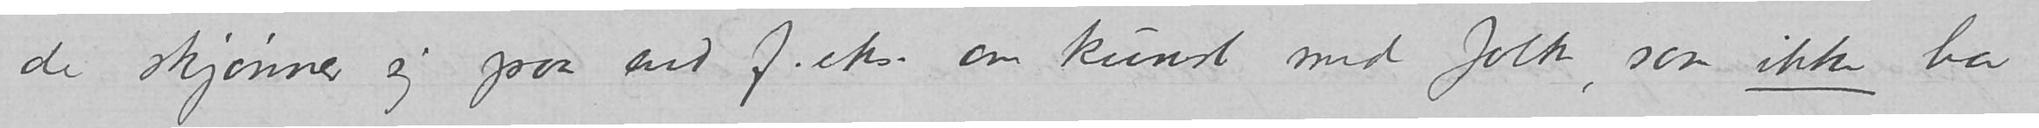de skjønner sig paa end f. eks. om kunst med folk, som ikke har
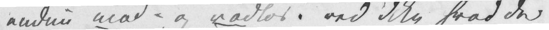endnu mod- og rådløs, ved ikke hvad der
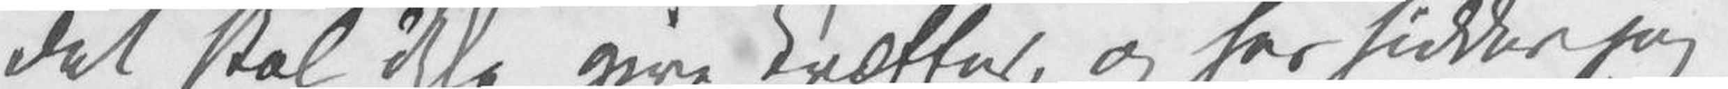det skal ikke give Kræfter, og her sidder jeg
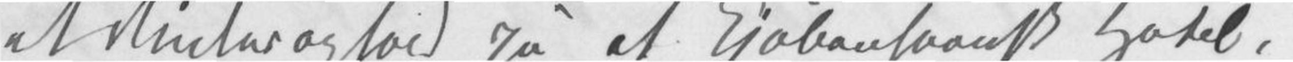et Vinterophold på et kjøbenhavnsk Hotel,
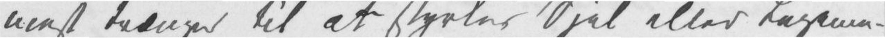mest trænger til at styrkes Sjel eller Legeme.
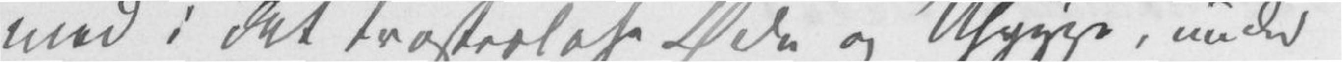med i det trøstesløse Øde og Uhygge, under
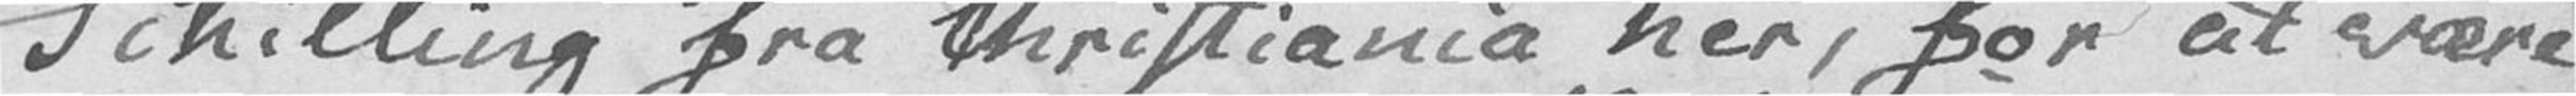Schilling fra Christiania her, for at være
